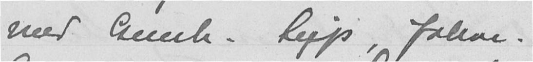med Gunh. Seip, Johan.
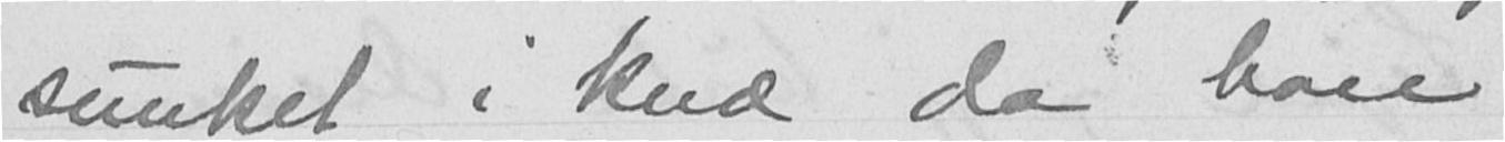sunket i knæ da han
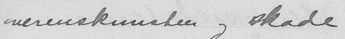overenskomsten og skade
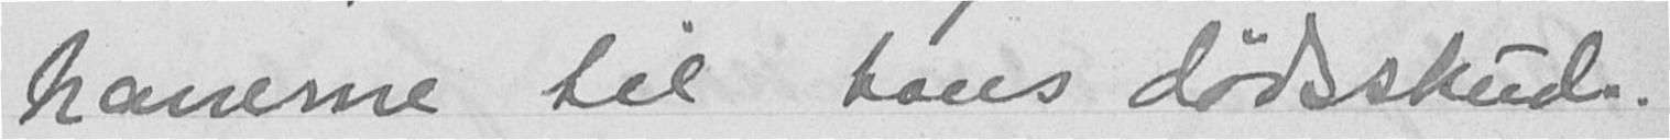hanerne til hans dødsskud.
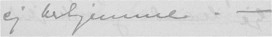sig herhjemme. -
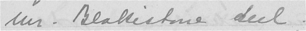mr. Blakistone anl.
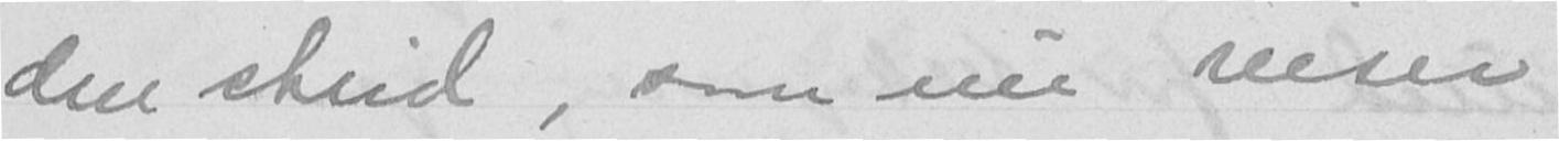den strid, som nu reiser
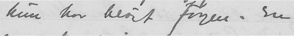hun har besøgt Jørgen. En
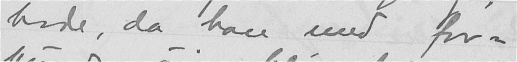havde, da han med for-
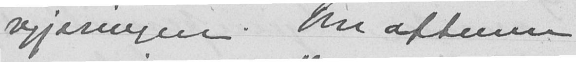regjeringen. Om aftenen
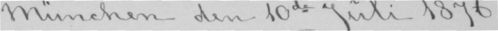München den 10de Juli 1876.
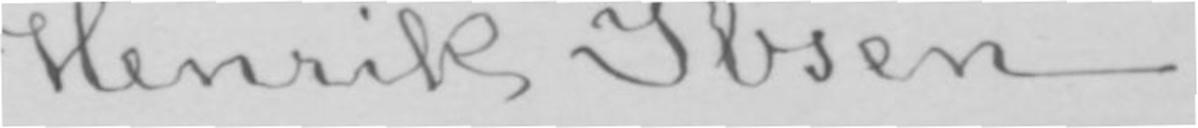Henrik Ibsen.
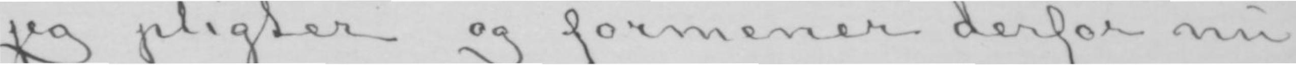jeg pligter og formener derfor nu
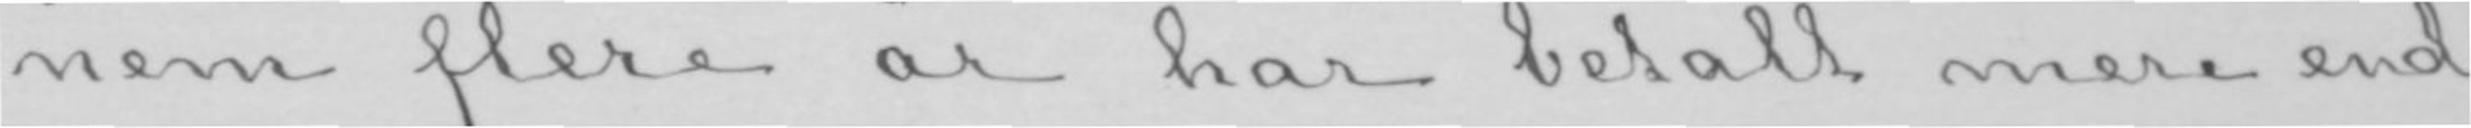nem flere år har betalt mere end
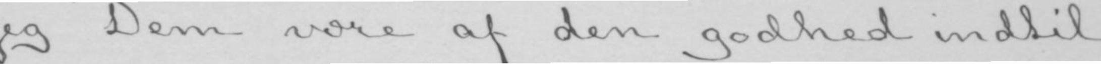jeg Dem være af den godhed indtil
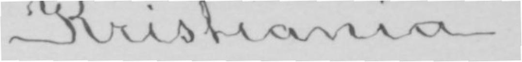Kristiania.
